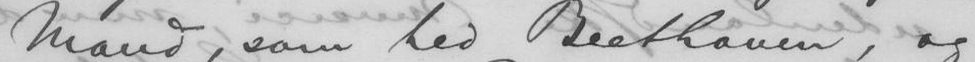Mand, som hed Beethoven, og
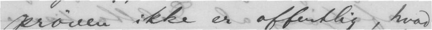prøven ikke er offentlig, hvad
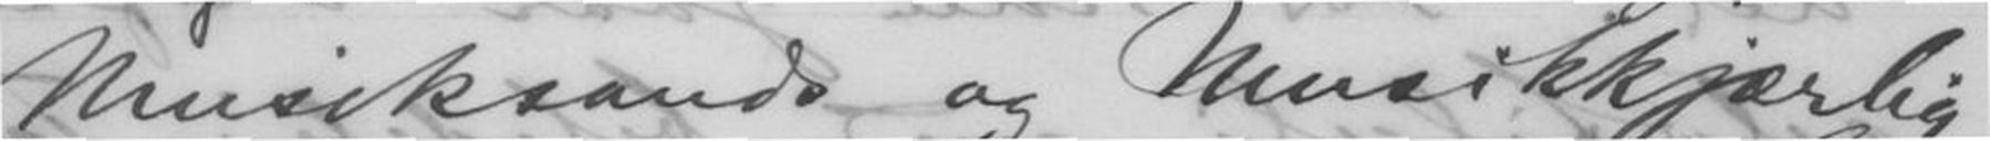Musiksands og Musikkjærlig-
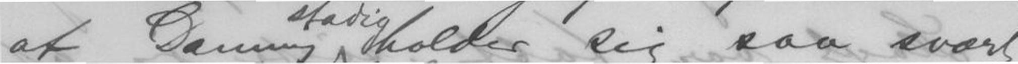at Danning holder sig saa svært
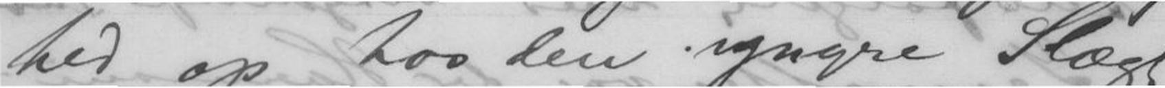hed op hos den yngre Slægt,
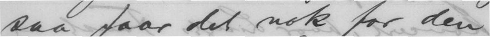saa faar det nok for den
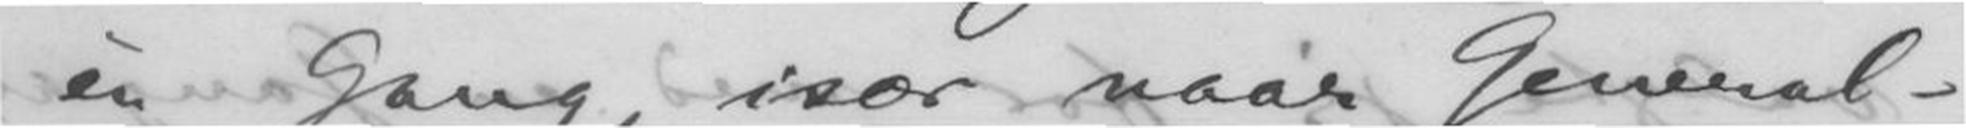én Gang, især naar General-
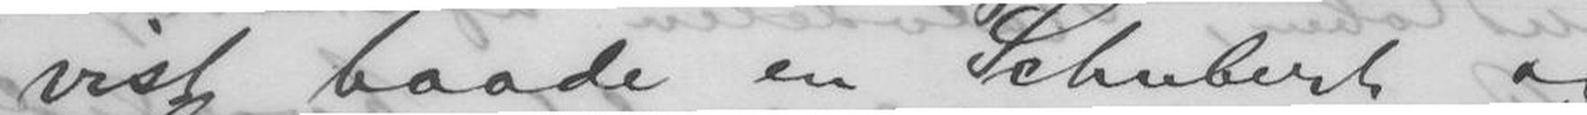vist baade en Schubert og
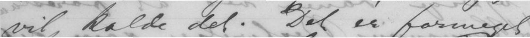vil kalde det. Det er formeget
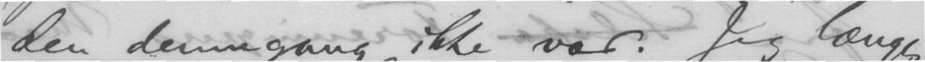den dennegang ikke var. Jeg længes
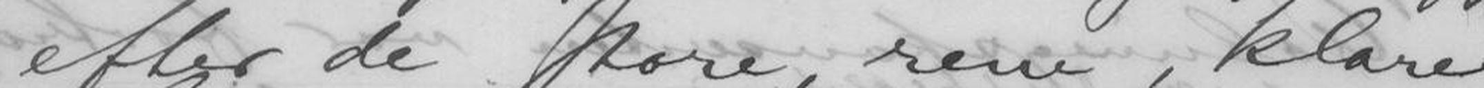efter de store, rene, klare
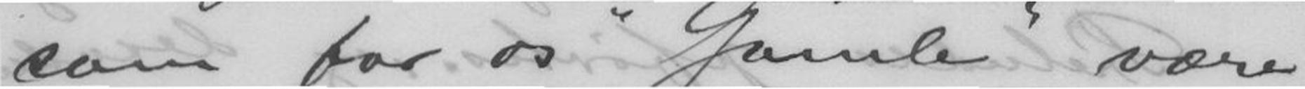som for os "Gamle" være
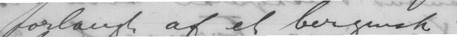forlangt af at bergensk
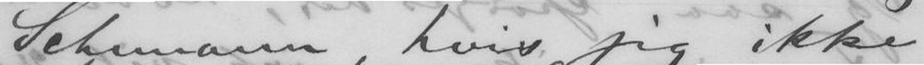Schumann, hvis jeg ikke
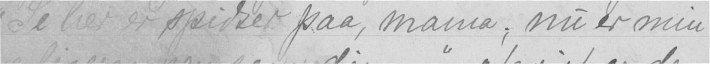"Se her er spidser paa, mama; nu er min
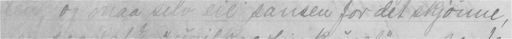den og maa selv eie sansen for det skjønne,
